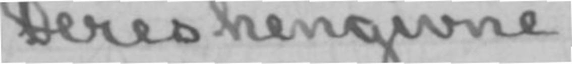Deres hengivne
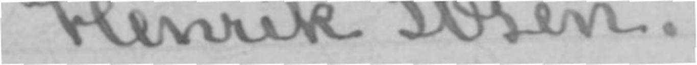Henrik Ibsen.
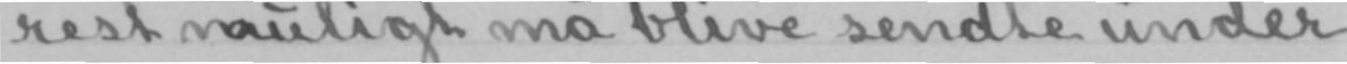rest muligt må blive sendte under
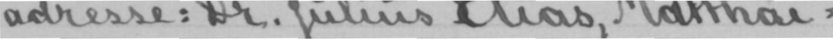adresse: Dr. Julius Elias, Matthäi-
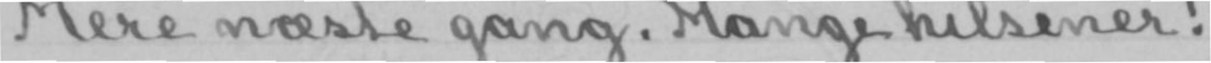Mere næste gang. Mange hilsener!
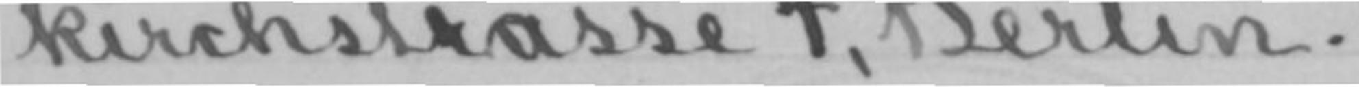kirchstrasse 4, Berlin.
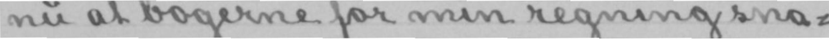nu at bøgerne for min regning sna-
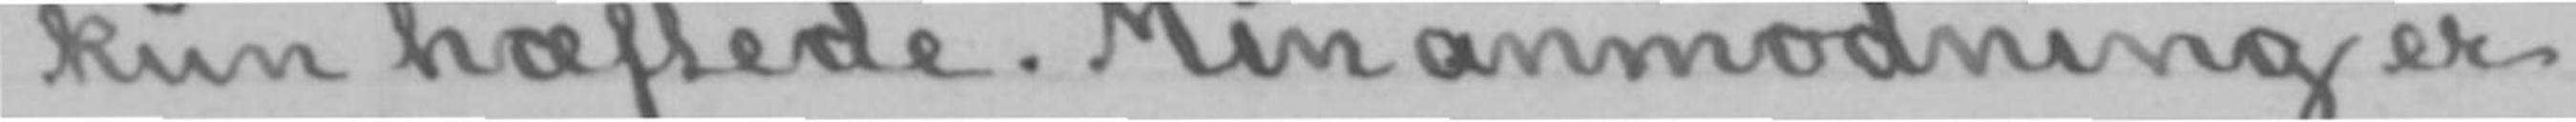kun hæftede. Min anmodning er
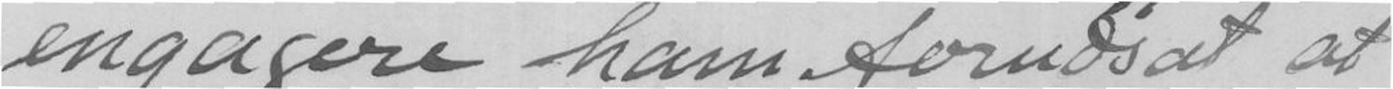engagere ham forudsat at
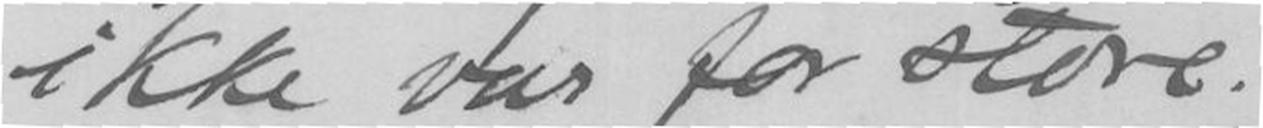ikke var for store.
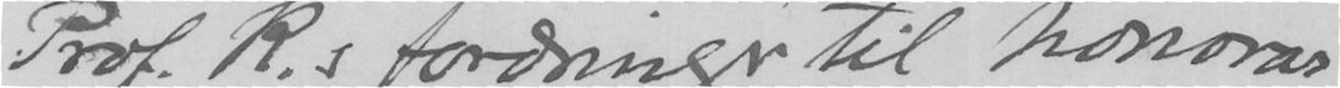Prof.R.s fordringer til honorar
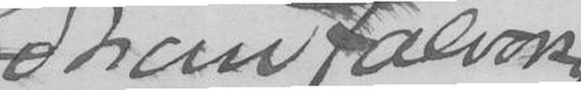Johan Halvorsen
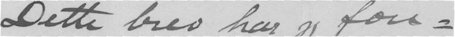Dette brev har jeg fore-
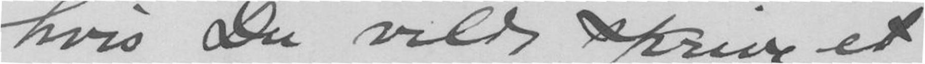hvis Du vilde skrive et
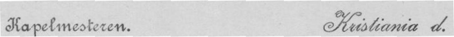Kapelmesteren. Kristiania d.
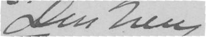Din heng
